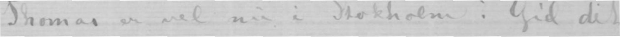Thomas er vel nu i Stokholm? Gid det
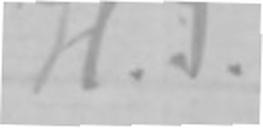H. I.
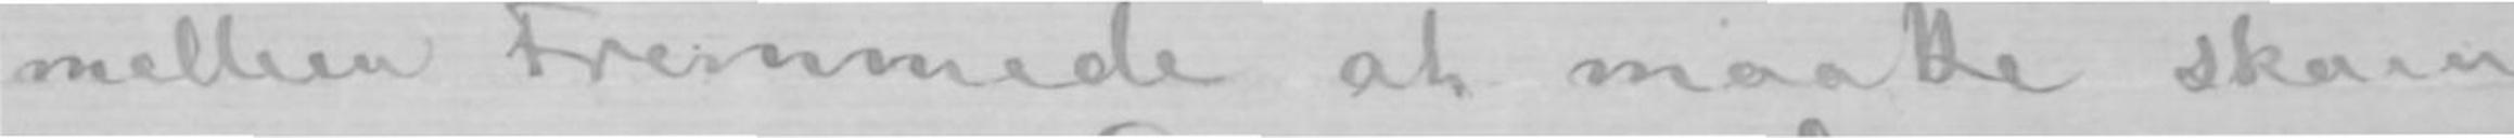i mellem Fremmede at maatte skam-
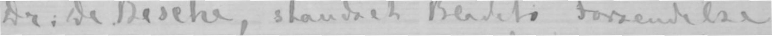Dr: De Besche, standset Bladets Forsendelse
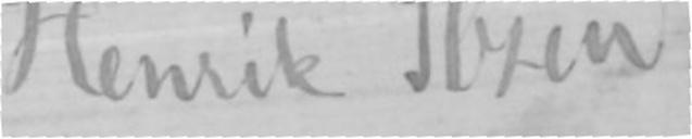Henrik Ibsen.
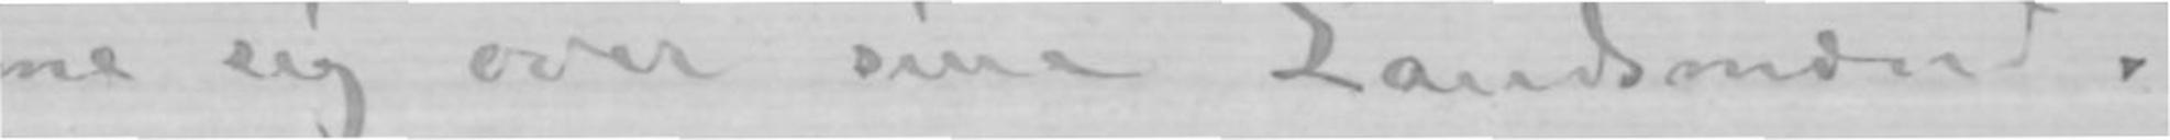me sig over sine Landsmænd.
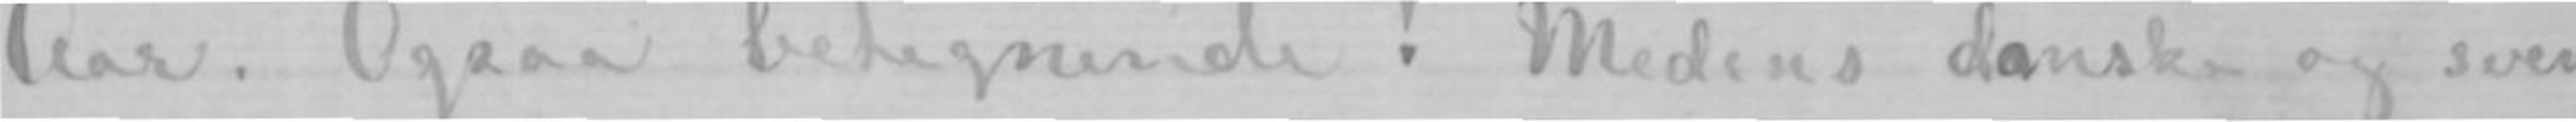Aar. Ogsaa betegnende! Medens danske og sven-
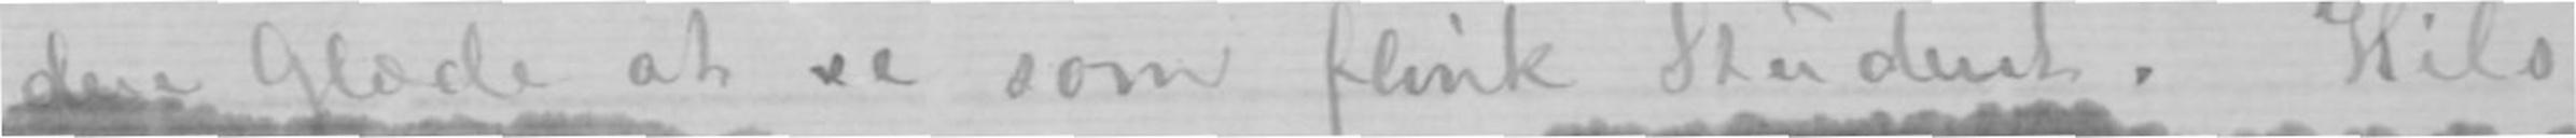den Glæde at se som flink Student. Hils
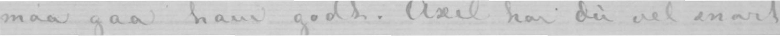maa gaa ham godt. Axel har Du vel snart
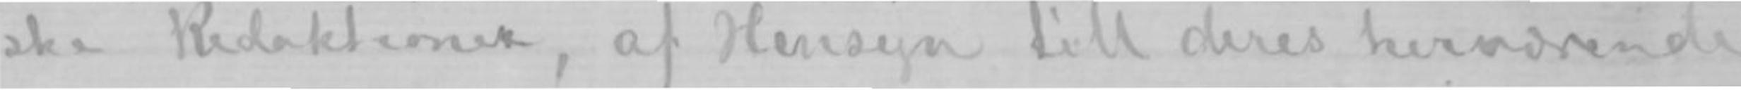ske Redaktioner, af Hensyn till deres herværende
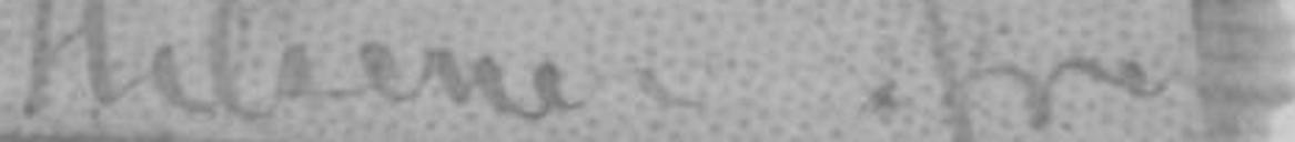Hilsener fra
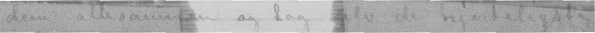dem allesammen og tag selv de hjerteligste
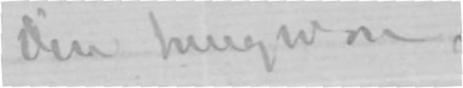Din hengivne
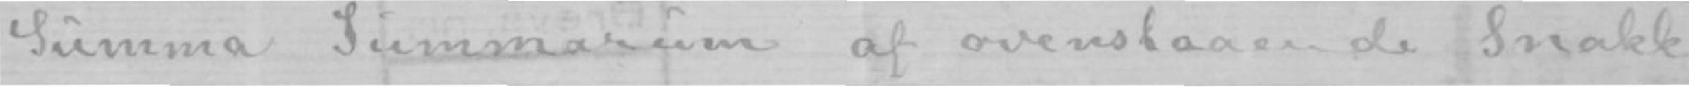Summa Summarum af ovenstaaende Snakk
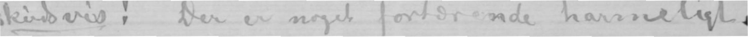skudsvis! Der er noget fortærende harmeligt
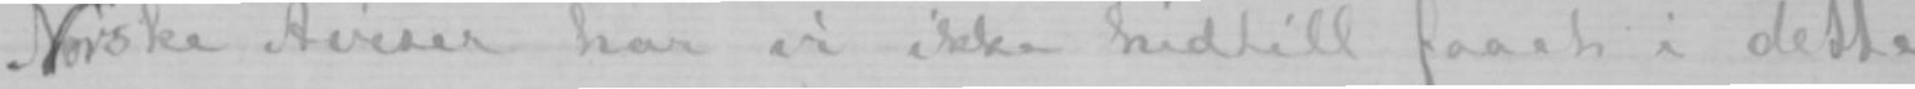Norske Aviser har vi ikke hidtill faaet i dette
